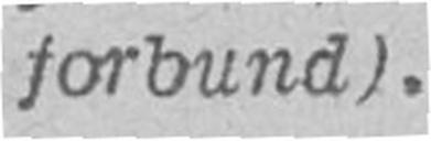forbund).
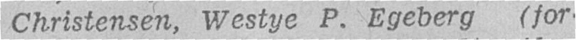Christensen, Westye P. Egeberg (for-
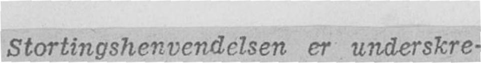Stortingshenvendelsen er underskre-
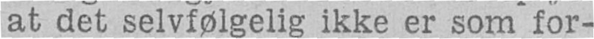at det selvfølgelig ikke er som for-
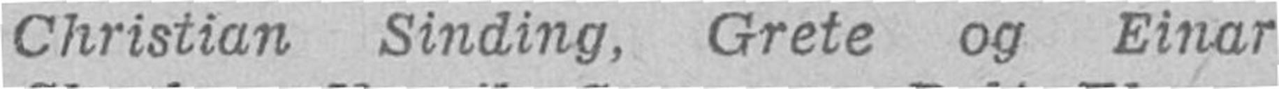Christian Sinding, Grete og Einar
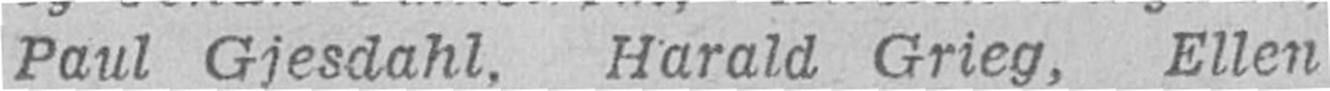Paul Gjesdahl, Harald Grieg, Ellen
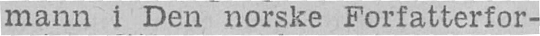mann i Den norske Forfatterfor-
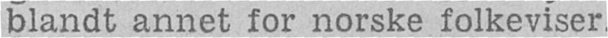blandt annet for norske folkeviser.
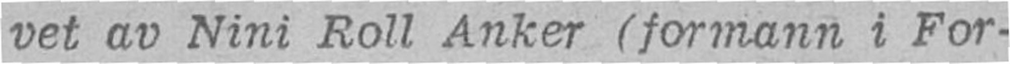vet av Nini Roll Anker (formann i For-
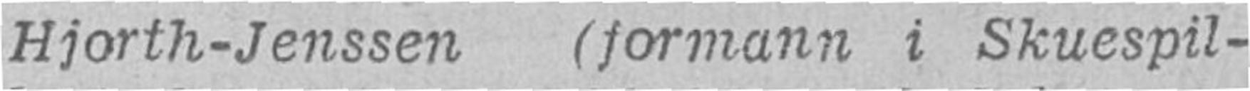Hjorth-Jenssen (formann i Skuespil-
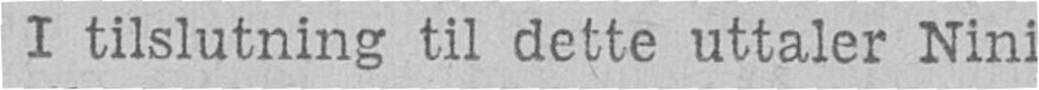I tilslutning til dette uttaler Nini
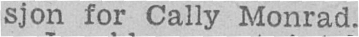sjon for Cally Monrad.
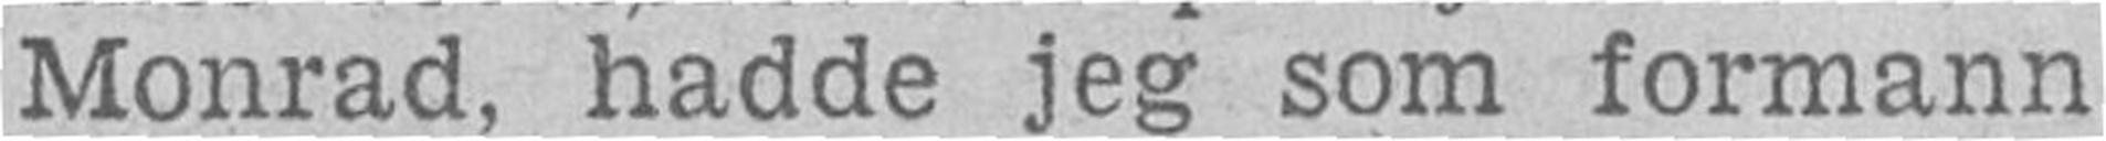Monrad, hadde jeg som formann
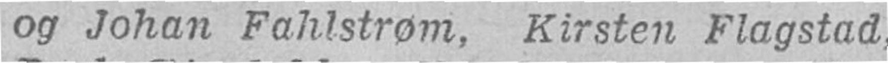og Johan Fahlstrøm, Kirsten Flagstad
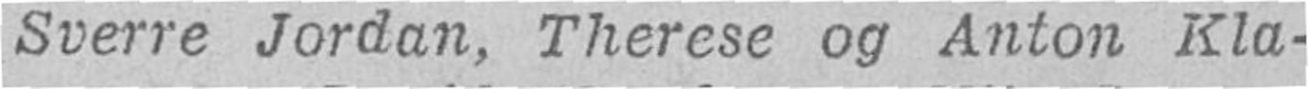Sverre Jordan, Therese og Anton Kla-
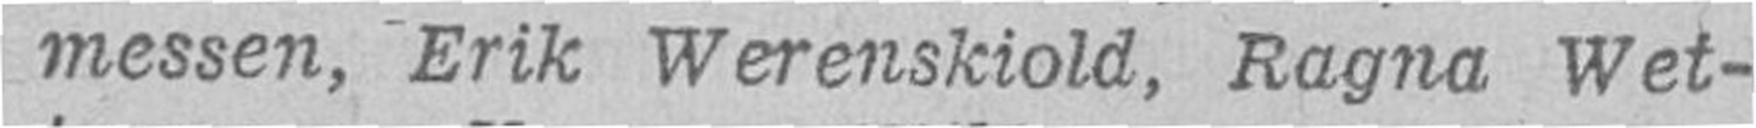messen, Erik Werenskiold, Ragna Wet-
